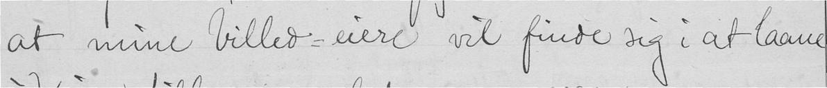at mine billed-eiere vil finde sig i at laane
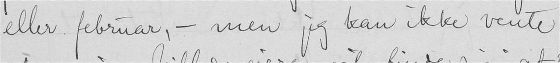eller februar, - men jeg kan ikke vente
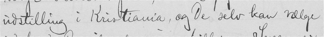udstilling i Kristiania, og De selv kan vælge
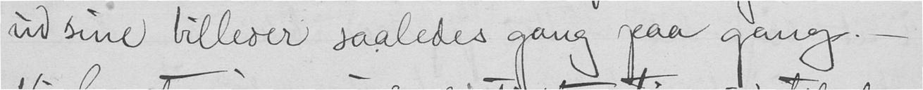ud sine billeder saaledes gang paa gang. -
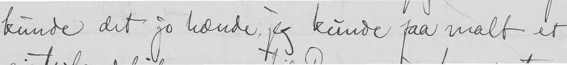kunde det jo hænde, jeg kunde faa malt et
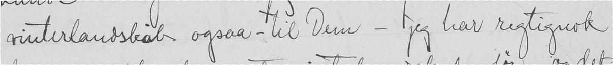vinterlandskab ogsaa - til Dem - jeg har rigtignok
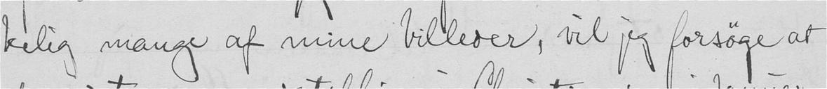kelig mange af mine billeder, vil jeg forsøge at
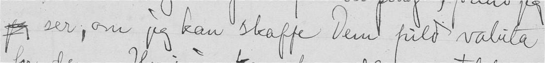ser, om jeg kan skaffe Dem fuld valuta
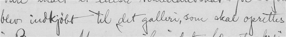blev indkjøbt til det galleri, som skal oprettes
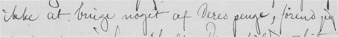ikke at bruge noget af Deres penge, førend jeg
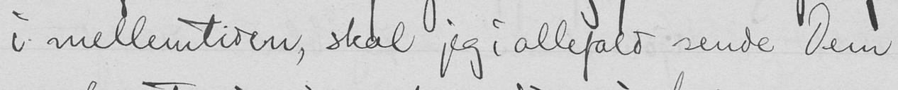i mellemtiden, skal jeg iallefald sende Dem
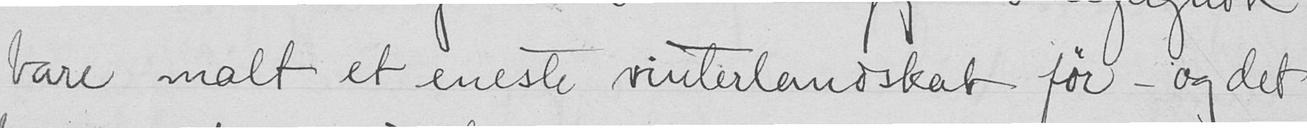bare malt et eneste vinterlandskab før - og det
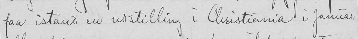faa istand en udstilling i Chistiania i januar
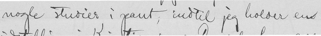nogle studier i pant, indtil jeg holder en
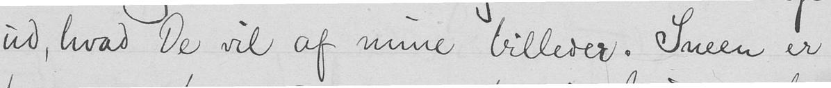ud, hvad De vil af mine billeder. Sneen er
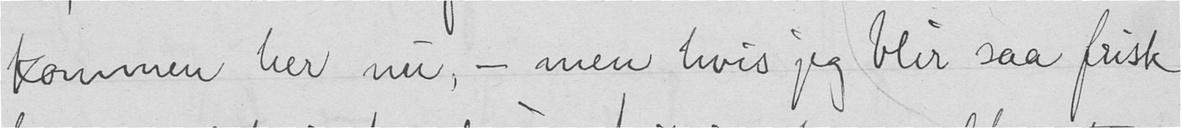kommen her nu, - men hvis jeg blir saa frisk
